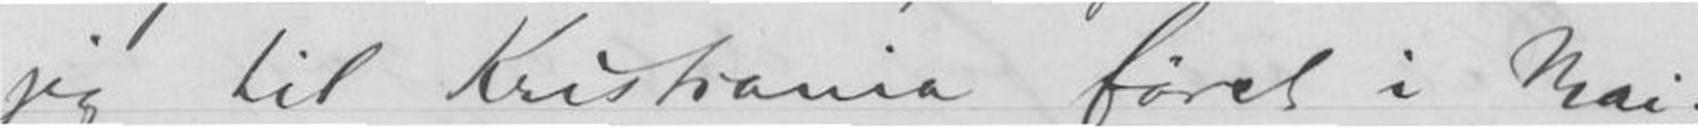jeg til Kristiania først i Mai.
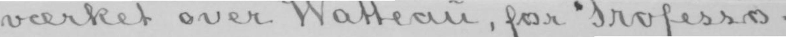værket over Watteau, for «Professo-
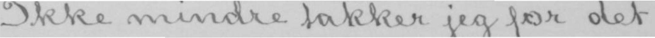Ikke mindre takker jeg for det
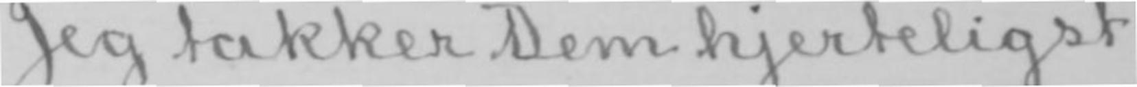Jeg takker Dem hjerteligst
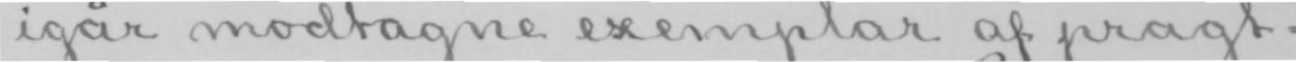igår modtagne exemplar af pragt-
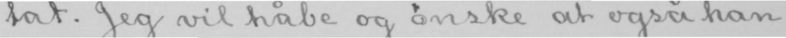tat. Jeg vil håbe og ønske at også han
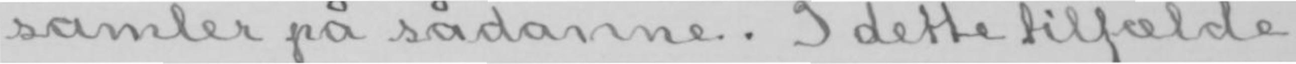samler på sådanne. I dette tilfælde
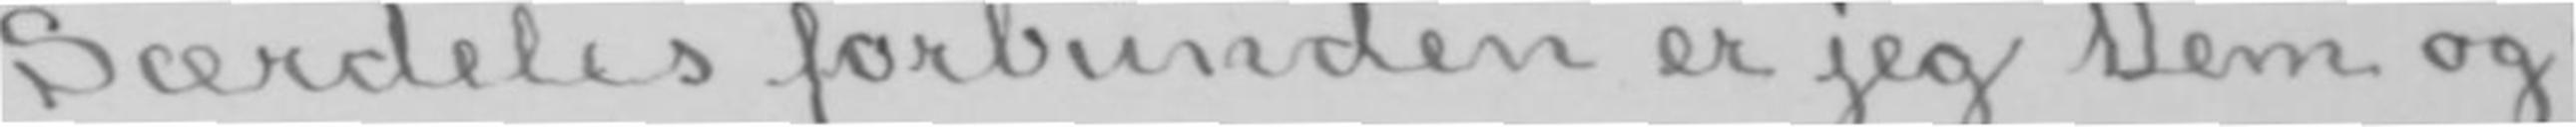Særdeles forbunden er jeg Dem og-
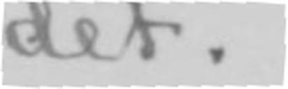det.
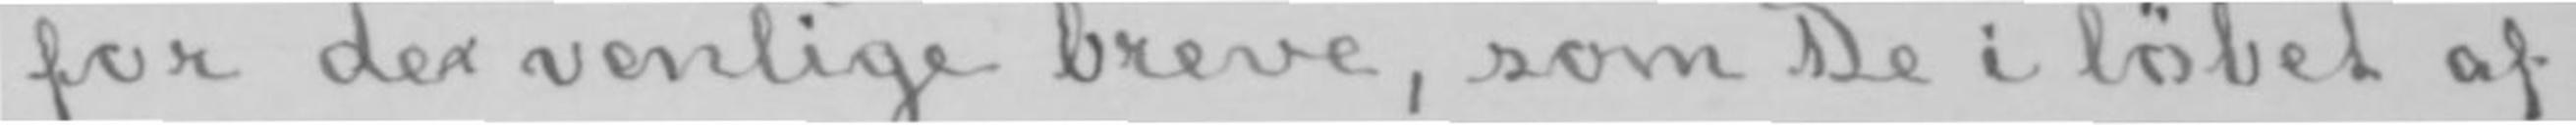for de venlige breve, som De i løbet af
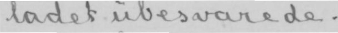ladet ubesvarede.
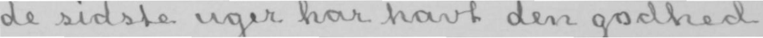de sidste uger har havt den godhed
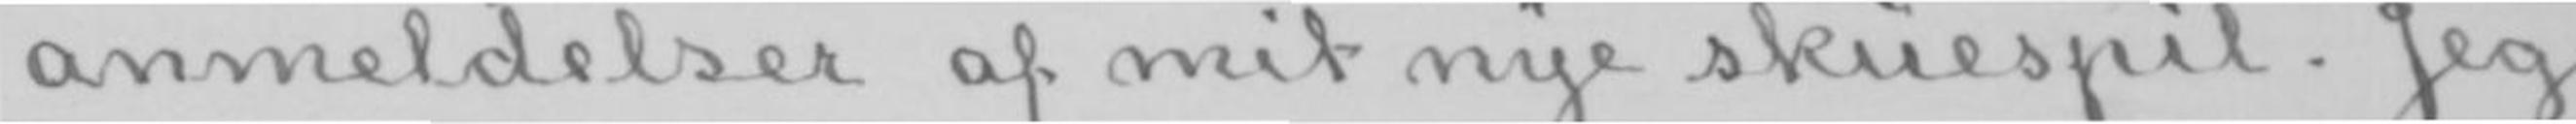anmeldelser af mit nye skuespil. Jeg
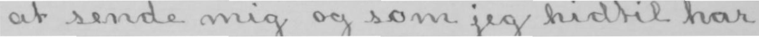at sende mig og som jeg hidtil har
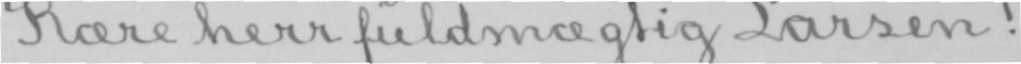Kære herr fuldmægtig Larsen!
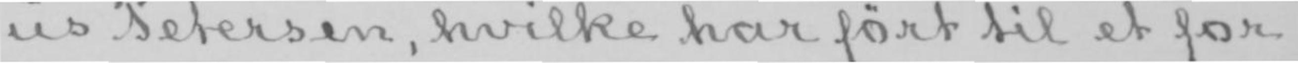us Petersen, hvilke har ført til et for
